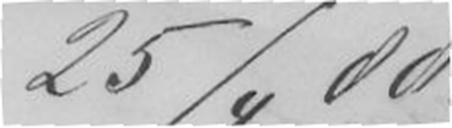25/4 88
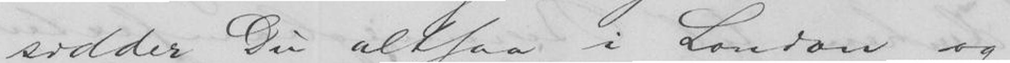sidder Du altsaa i London og
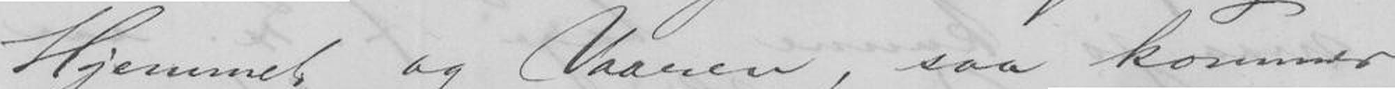Hjemmet og Vaaren, saa kommer
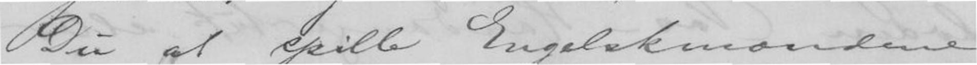Du at spille Engelskmændene
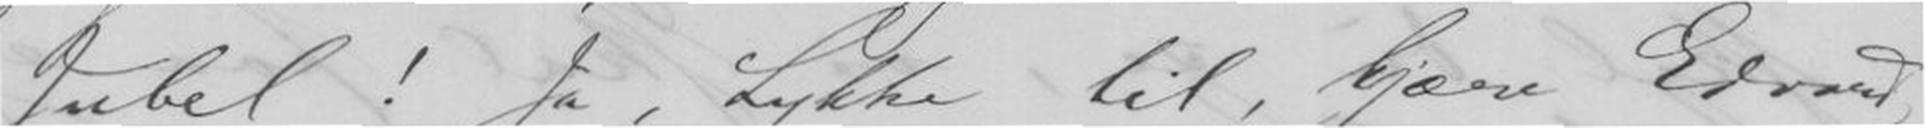Jubel! Ja, Lykke til, kjære Edvard,
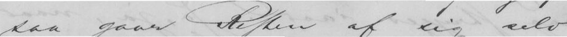saa gaar Resten af sig selv.
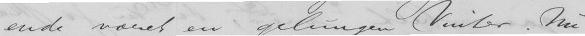ende været en gelungen Vinter. Nu
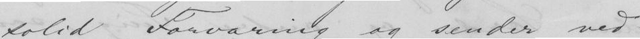solid Forvaring og sender ved-
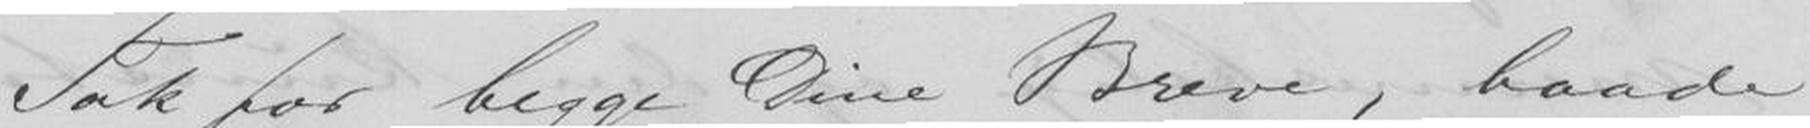Tak for begge Dine Breve, baade
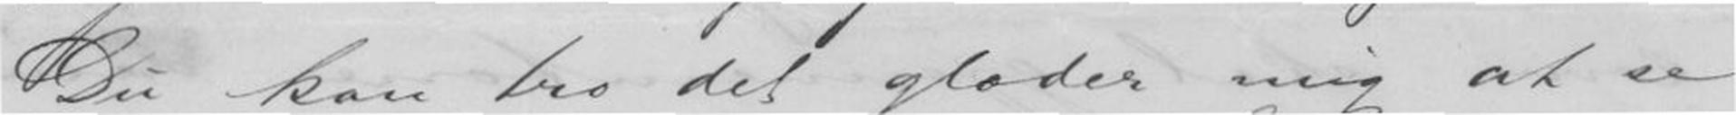Du kan tro det glæder mig at se
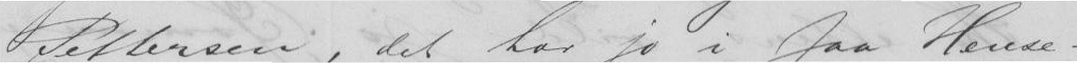Pettersen, det har jo i saa Hense-
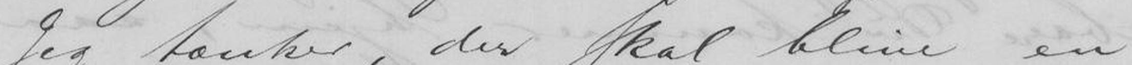Jeg tænker, der skal blive en
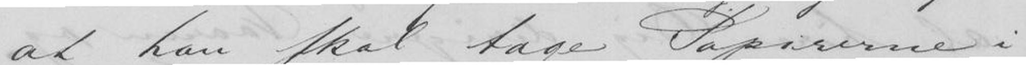at han skal tage Papirne i
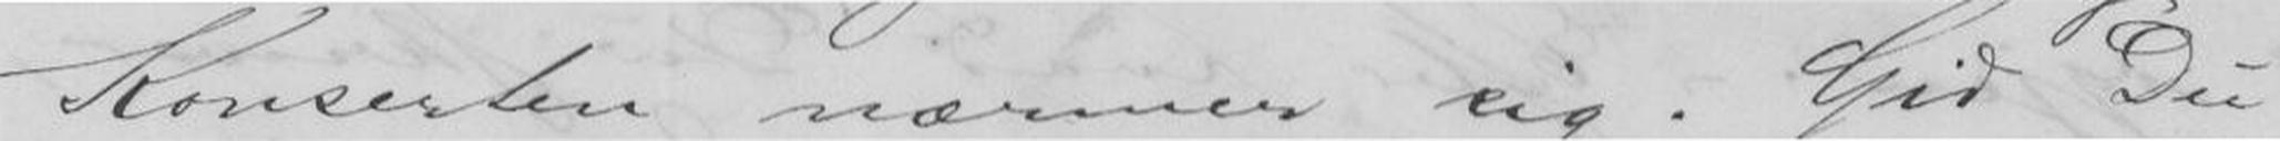Konserten nærmer sig. Gid Du
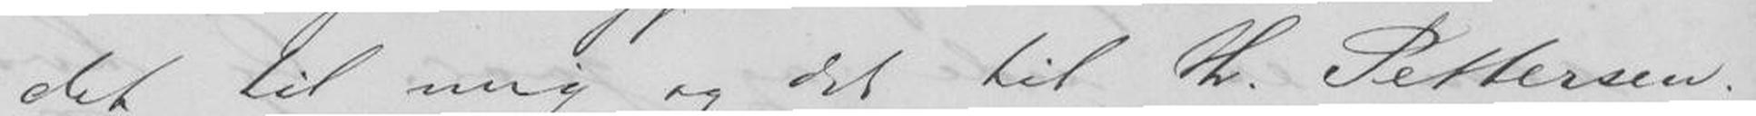det til mig og det til Hr. Pettersen.
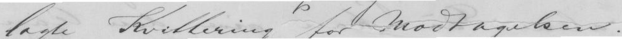lagte Kvittering for Modtagelsen.
